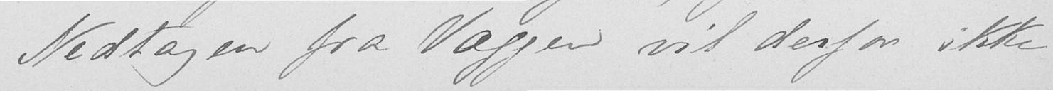Nedtagen fra Væggen vil derfor ikke
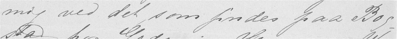mig ved det, som findes paa Bog-
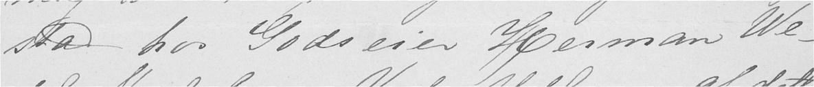stad hos Godseier Herman We-
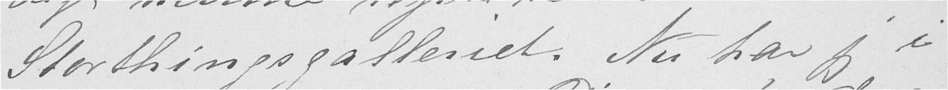Storthingsgalleriet. Nu har jeg i
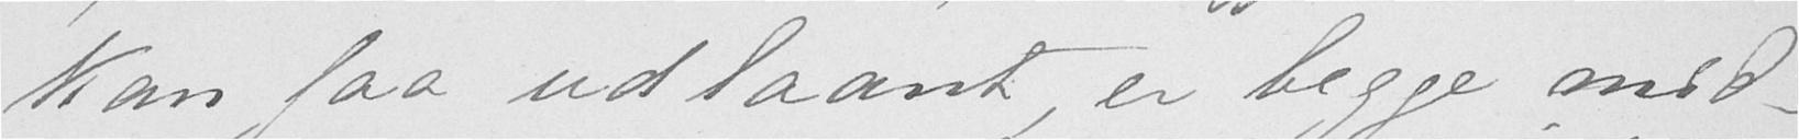kan faa udlaant er begge mid-
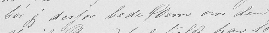tør jeg derfor bede Dem om den
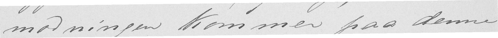modningen kommer paa denne
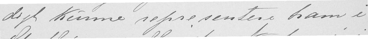digt kunne representere ham i
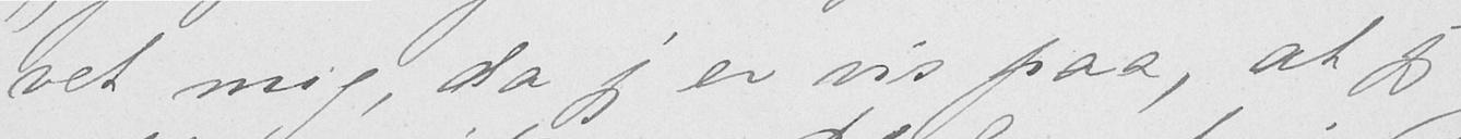vet mig, da jeg er vis paa, at jeg
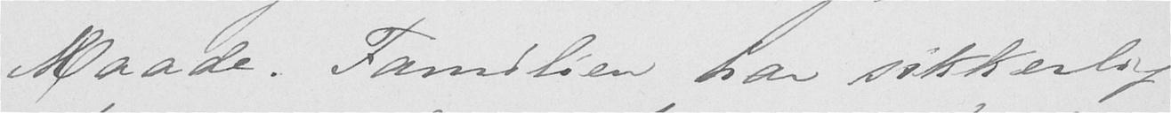Maade. Familien har sikkerlig
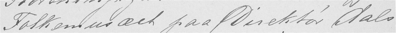Folkemusæet paa Direktør Aals
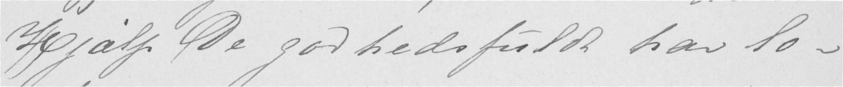Hjælp De godhedsfuldt har lo-
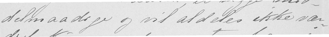delmaadige og vil aldeles ikke vær-
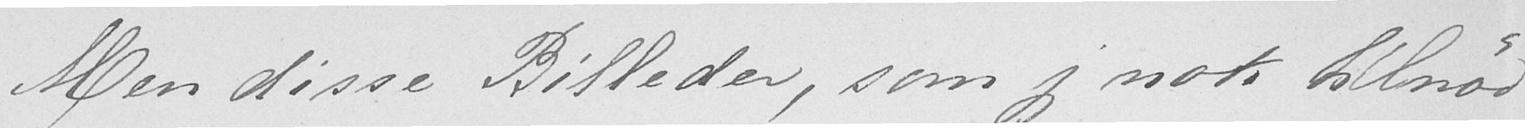Men disse Billeder, som jeg nok tilnød
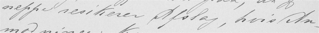neppe resikerer Afslag, hvis An-
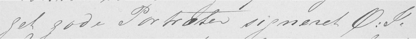get gode Portræter signeret O.J.
